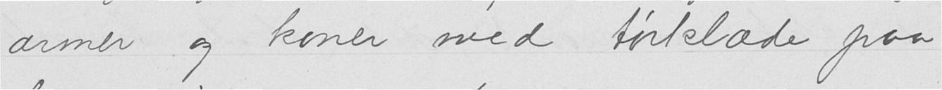armer og koner med tørklæde paa
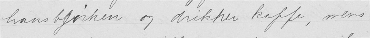hansbjørken og drikker kaffe, mens
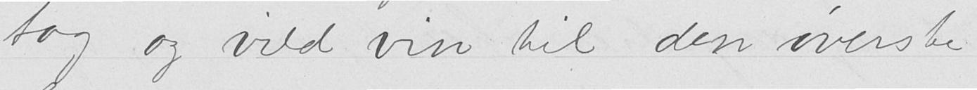tag og vild vin til den øverste
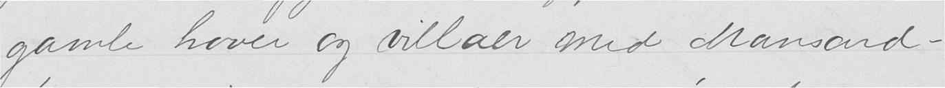gamle haver og villaer med Mansard-
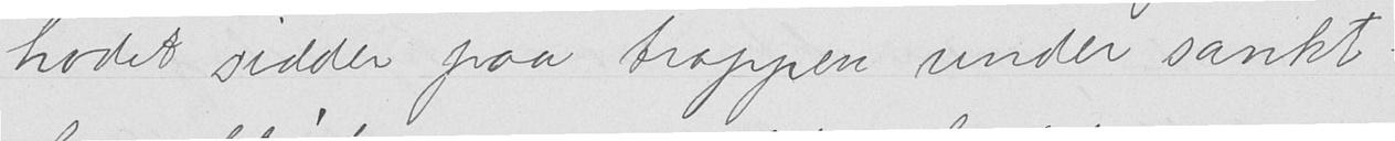hodet sidder paa trappen under sankt-
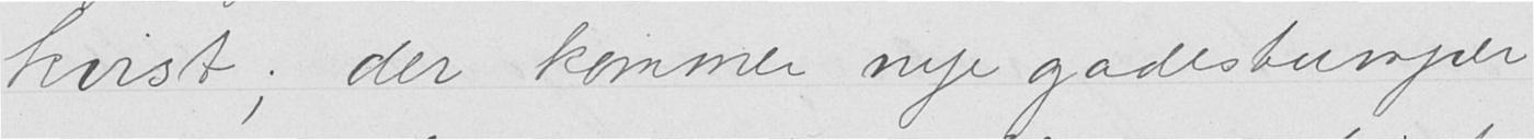kvist; der kommer nye gadestumper
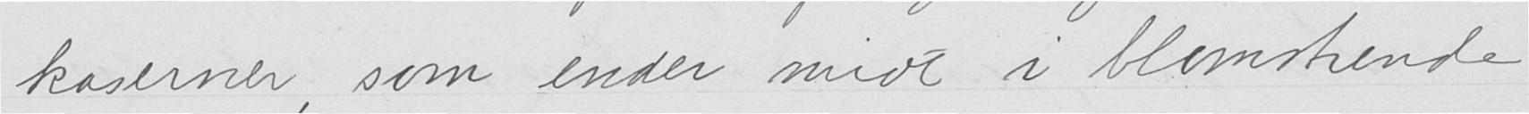kaserner, som ender midt i blomstrende
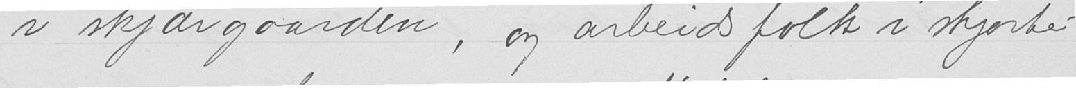i skjærgaarden, og arbeidsfolk i skjorte-
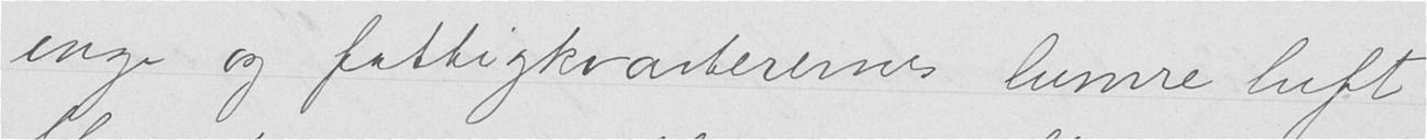enge og fattigkvarterernes lumre luft
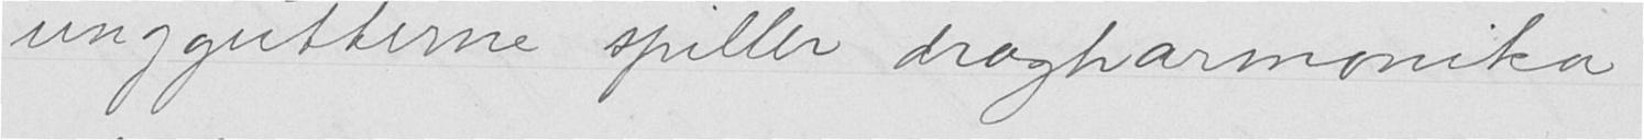unggutterne spiller dragharmonika
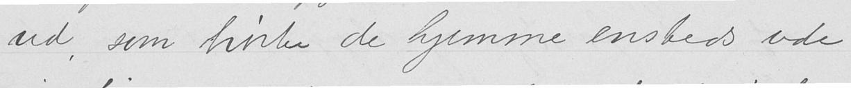ud, som hørte de hjemme ensteds ude
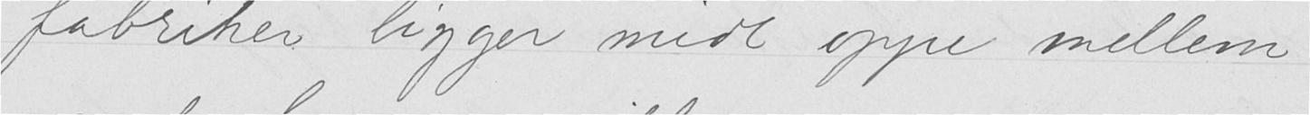fabriker ligger midt oppe mellem
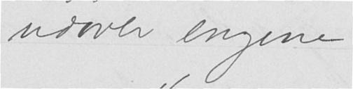udover engene.
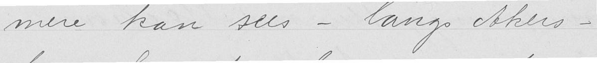mere kan sees - langs Aker-
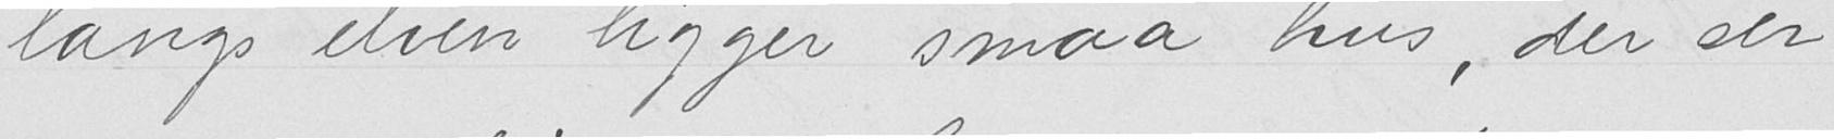langs elven ligger smaa hus, der ser
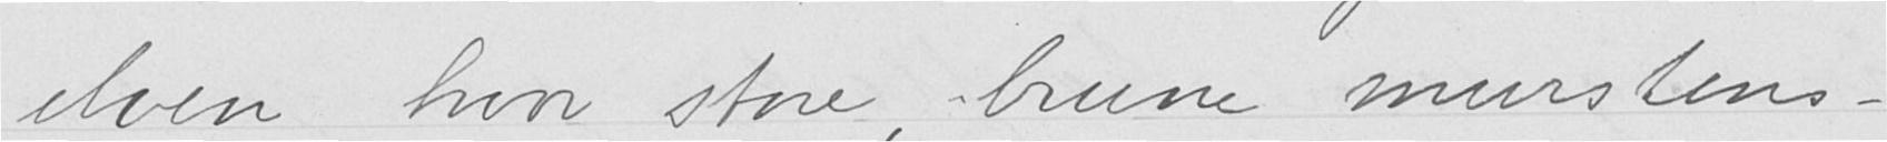selven, hvor store, brune murstens-
